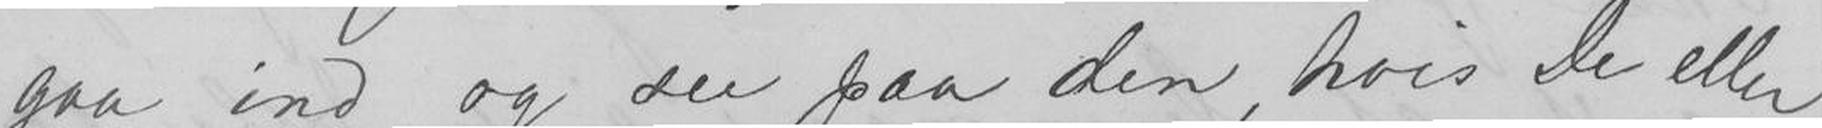gaa ind og see paa den, hvis De eller
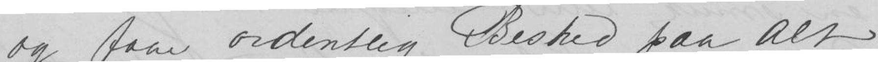og faae ordentlig Besked paa Alt,
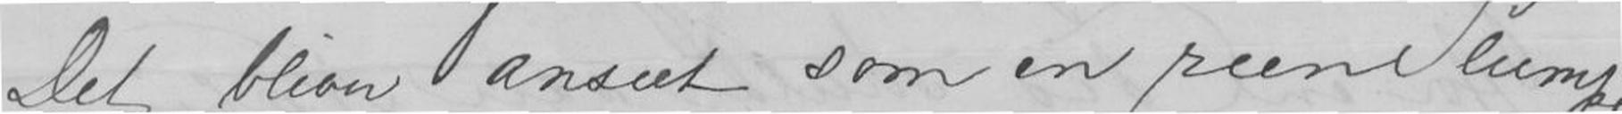Det bliver anseet som en reen Slumpe-
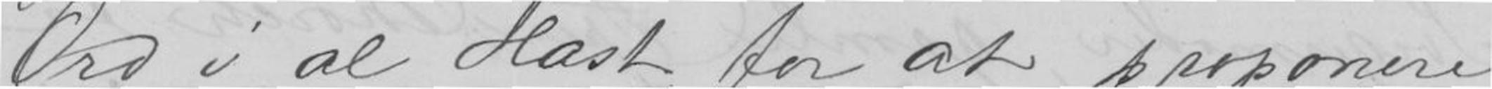Ord i al Hast, for at proponere
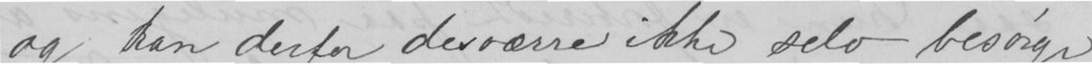og kan derfor desværre ikke selv besørge
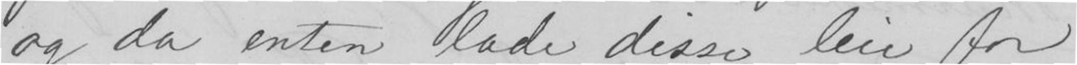og da enten lade disse leie for
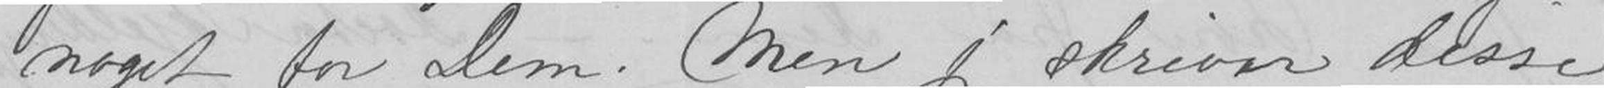noget for Dem. Men jeg skriver disse
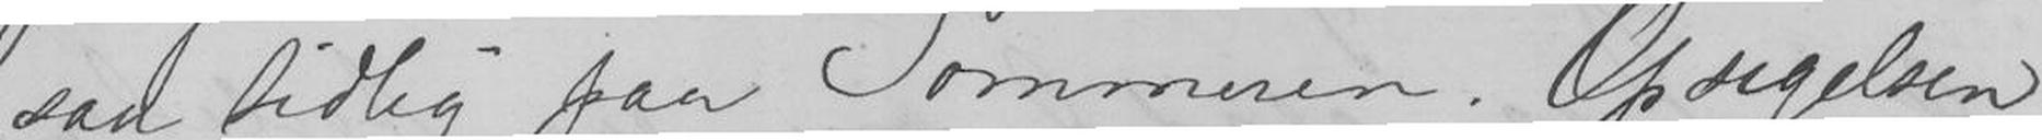saa tidlig paa Sommeren. Opsigelsen
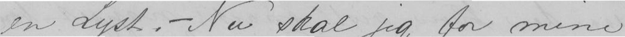en Lyst, - Nu skal jeg for mine
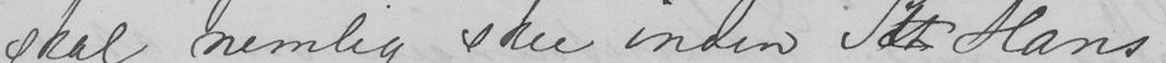skal nemlig skee inden Sth Hans.
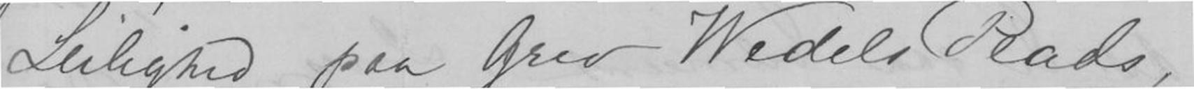Leilighed paa Grev Wedels Plads,
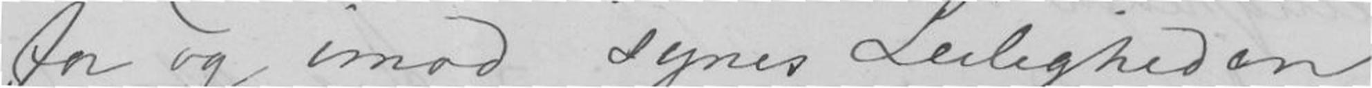for og imod synes Leiligheden
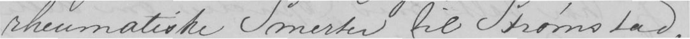rheumatiske Smerter til Strømstad,
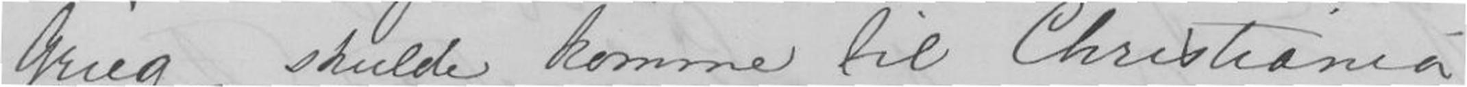Grieg skulde komme til Christiania
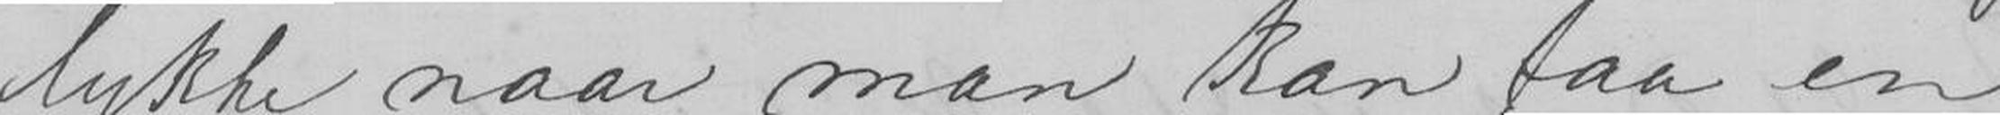lykke, naar man kan faa en
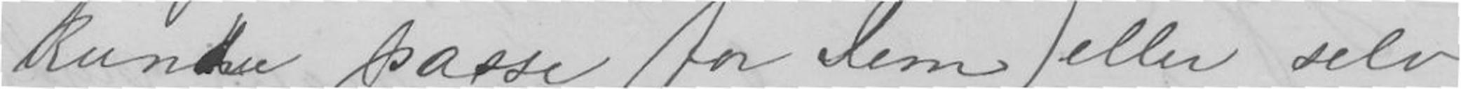kunde passe for Dem) eller selv
